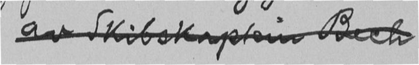av Skibskaptein Bech
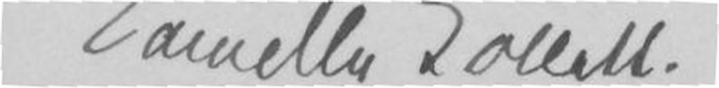Camilla Collett.
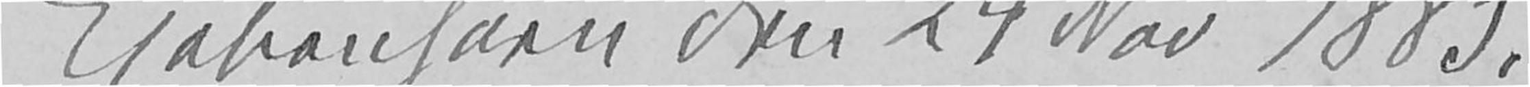Kjøbenhavn den 24 Nov 1883.
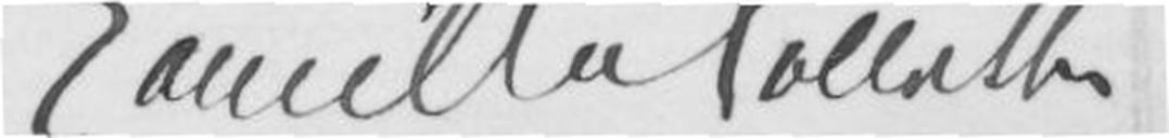Camilla Collett.
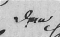Dem
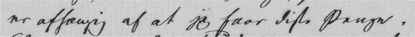er afhængig af at jeg faar disse Penge,
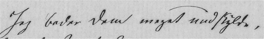Jeg beder Dem meget undskylde,
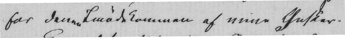for denne Imødekommen af mine Ønsker.
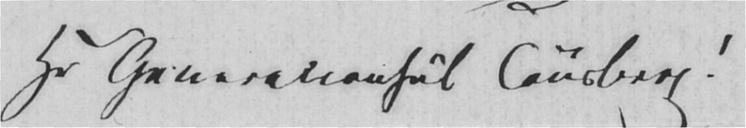Hr Generalconsul Tønsberg!
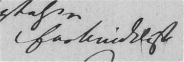forbindtligstTønsberg
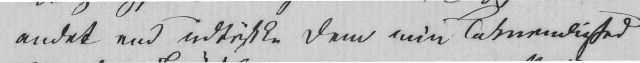andet end udtrykke Dem min Taknemlighed
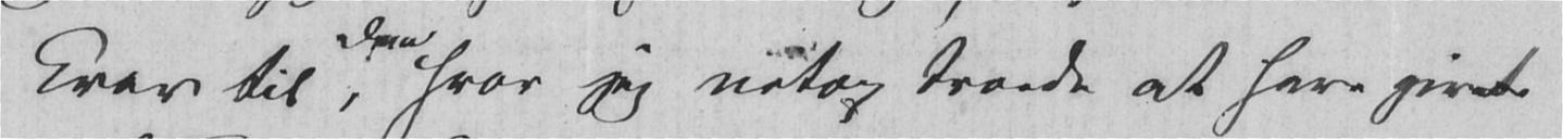Krav til, hvor jeg netop troede at have givet
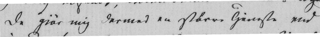De gjør mig dermed en større Tjeneste end
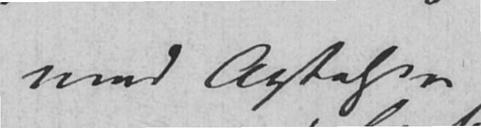med Agtelse
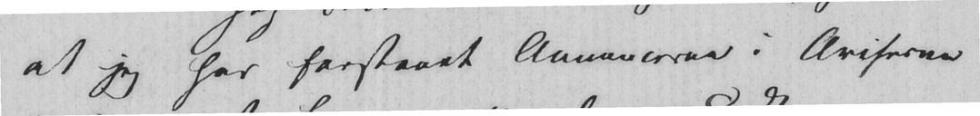at jeg har forstaaet Annoncerne i Aviserne
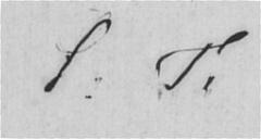S. T.
